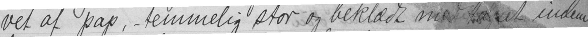vet af pap, - temmelig stor og beklædt med tapet indeni
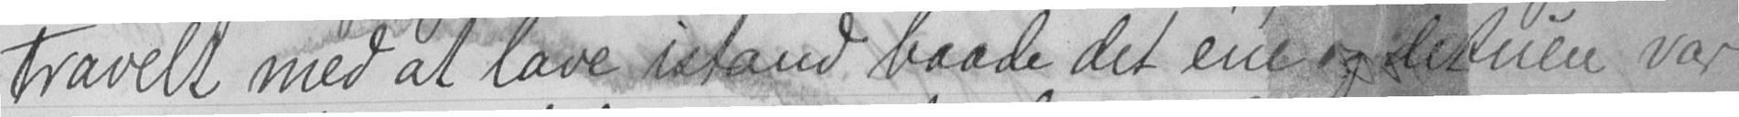travelt med at lave istand baade det ene og det tuen var
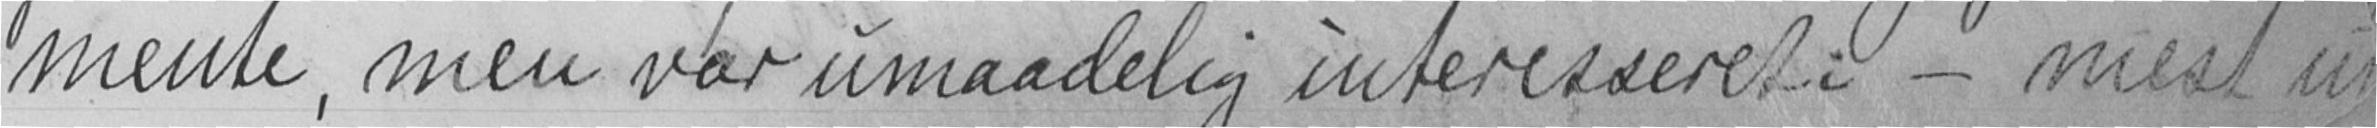mente, men var umaadelig interesseret i - mest u
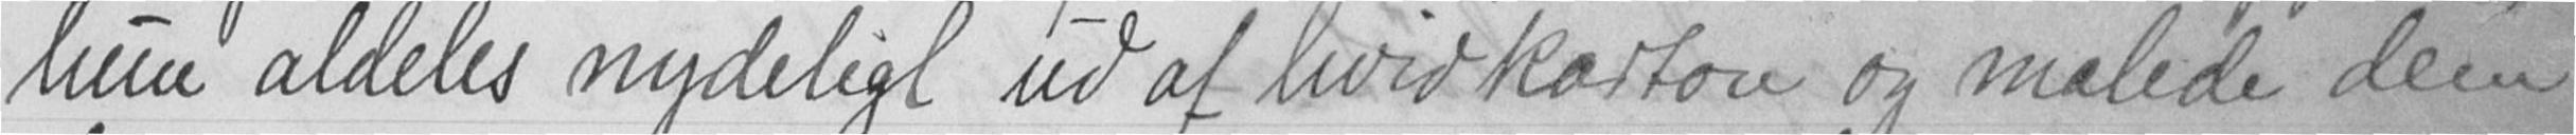hun aldeles nydeligt ud af hvid karton og malede dem
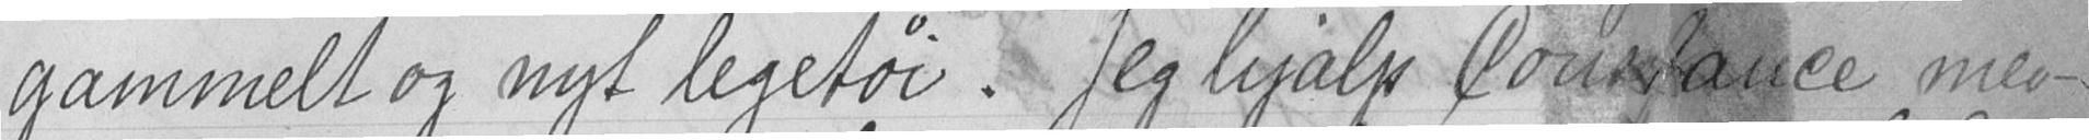gammelt og nyt legetøi. Jeg hjalp Constance med-
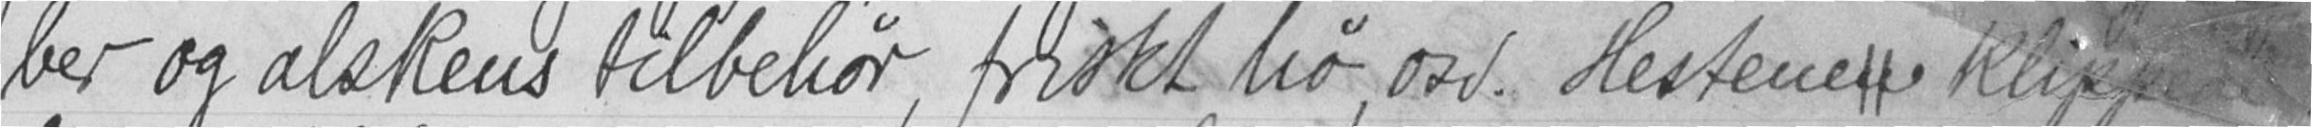ber og alskens tilbehør, friskt hø osv. Hestenen klippede
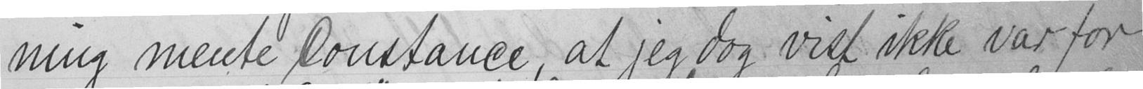ning mente Constance, at jeg dog vist ikke var for
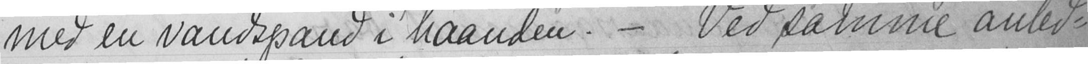med en vandspand i haanden. - Ved samme anled-
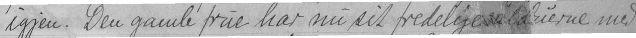igjen. Den gamle frue har nu sit fredelige uerne med
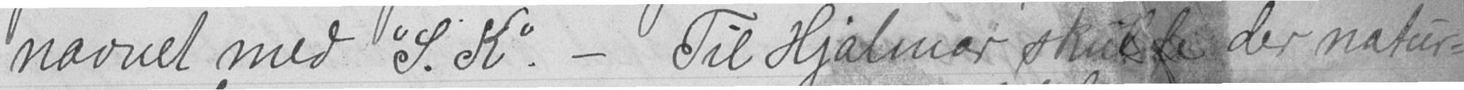navnet med "S. K". - Til Hjalmar skulde der natur-
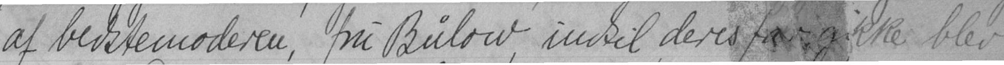af bedstemoderen, fru Bulow, indtil deres far g ikke blev
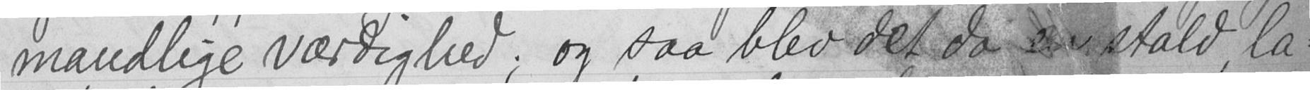mandlige værdighed; og saa blev det da en stald, la-
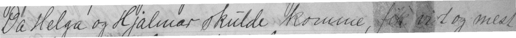Da Helga og Hjalmar skulde komme, fik vi  og mest
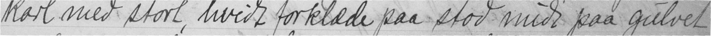karl med stort, hvidt forklæde paa stod midt paa gulvet
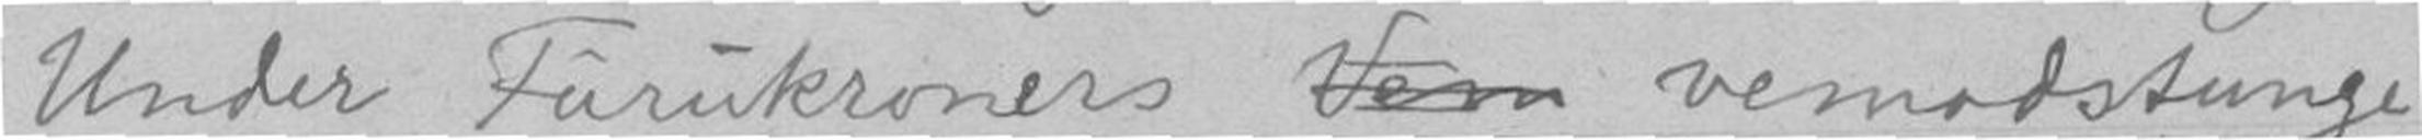Under Furukroners Vem vemodstunge
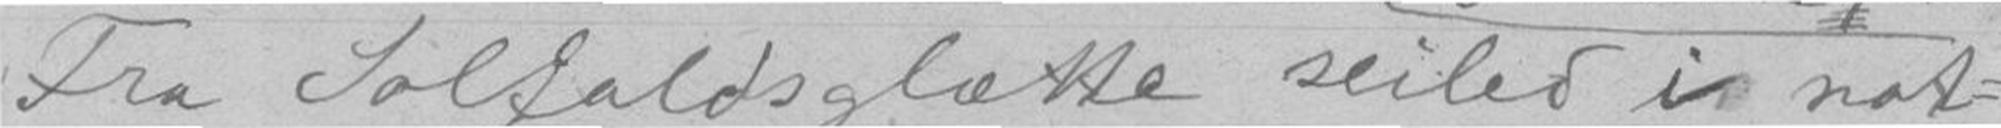Fra Solfaldsglætte seiled i nat-
